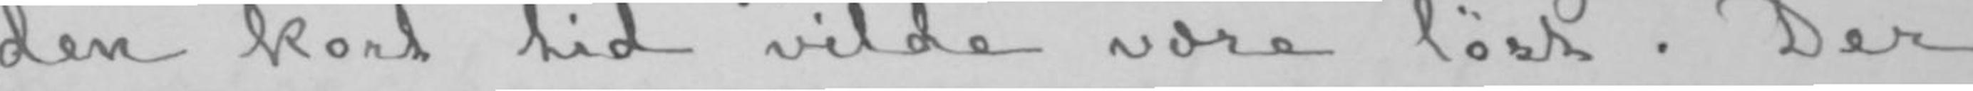den kort tid vilde være løst. Der
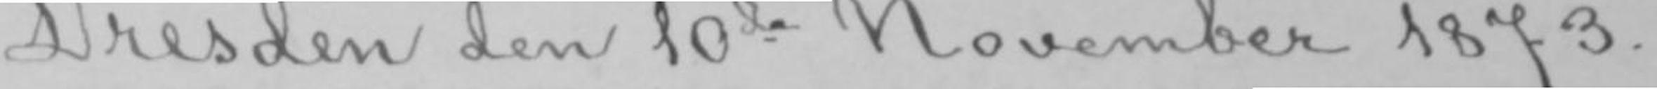Dresden den 10de November 1873.-
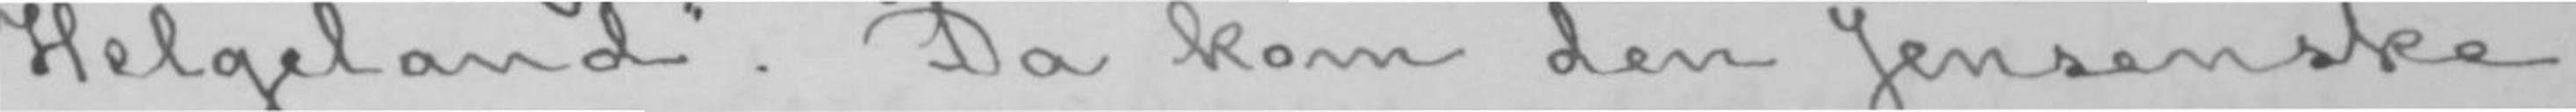Helgeland». Da kom den Jensenske
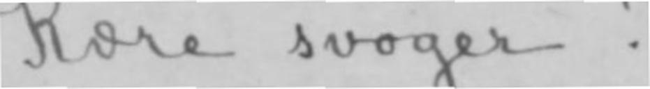Kære svoger!
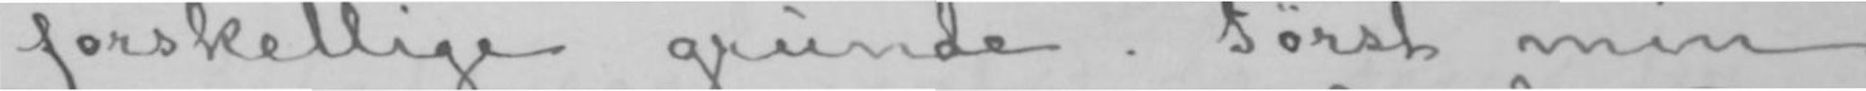jeg forskellige grunde. Først min
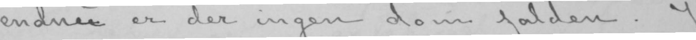endnu er der ingen dom falden. Jeg
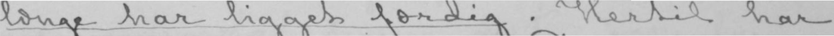længe har ligget færdig. Hertil har
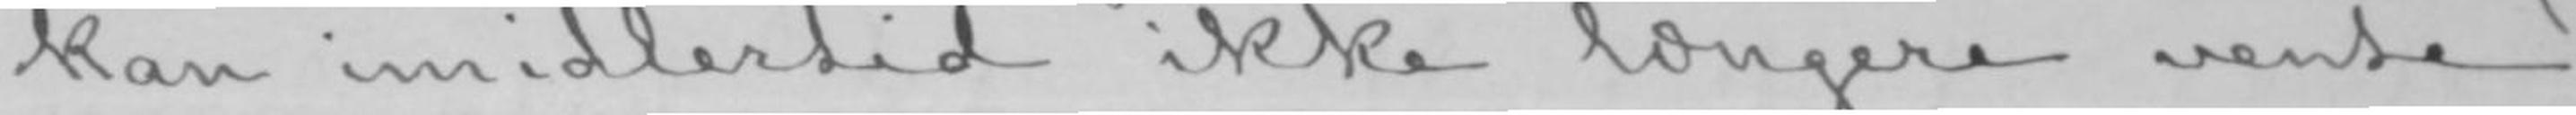kan imidlertid ikke længere vente
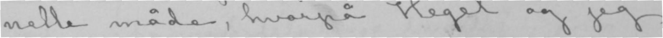nelle måde, hvorpå Hegel og jeg
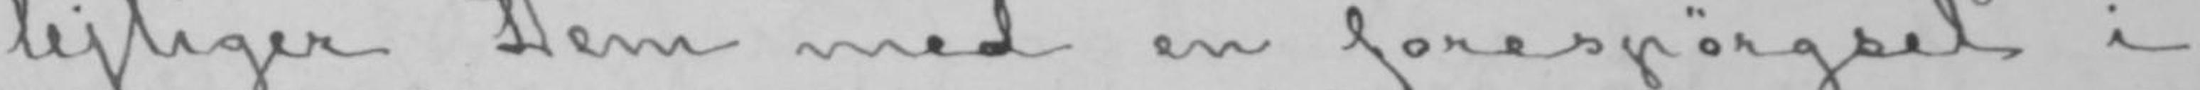lejliger Dem med en forespørgsel i
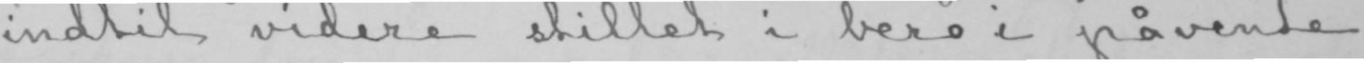indtil videre stillet i bero i påvente
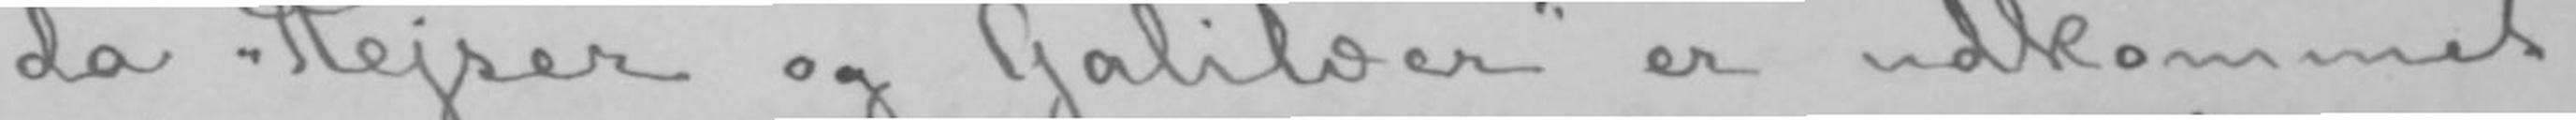da «Kejser og Galilæer» er udkommet.
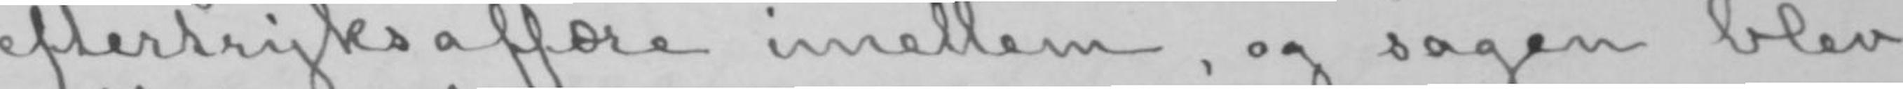eftertryksaffære imellem, og sagen blev
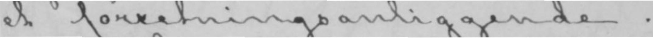et forretningsanliggende.
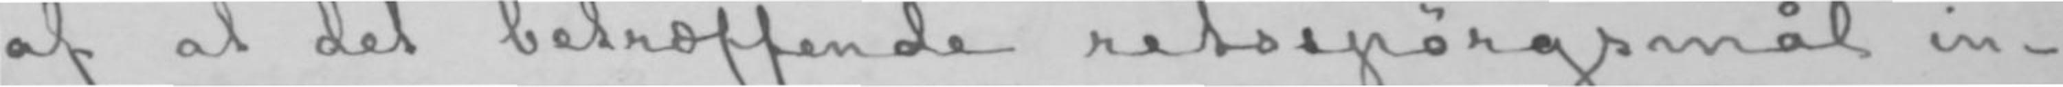af at det betræffende retsspørgsmål in-
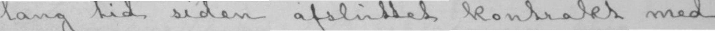lang tid siden afsluttet kontrakt med
